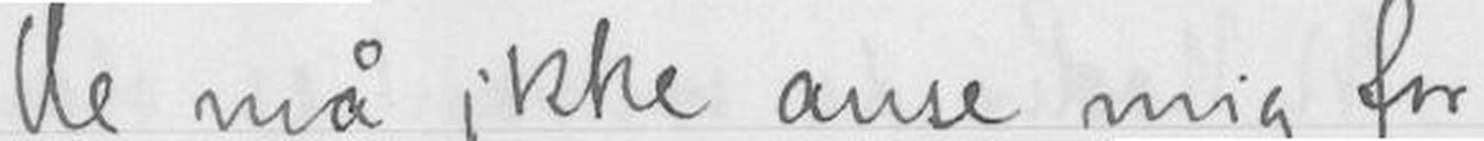De må ikke anse mig for
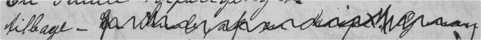tilbage -
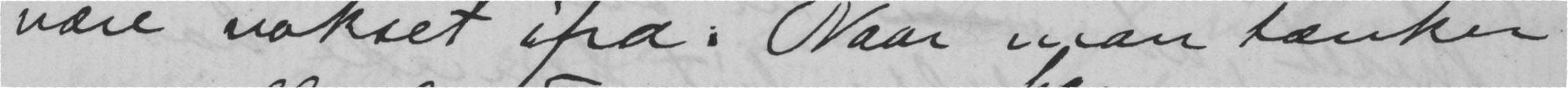være vokset ifra: Naar man tænker
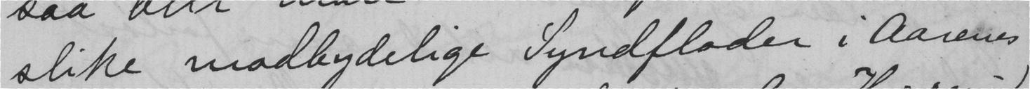slike modbydelige Syndfloder i Aarenes
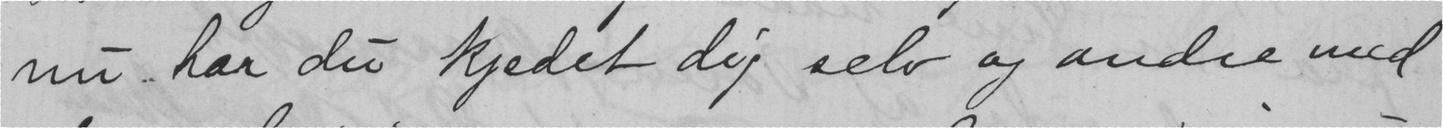nu har du kjedet dig selv og andre med
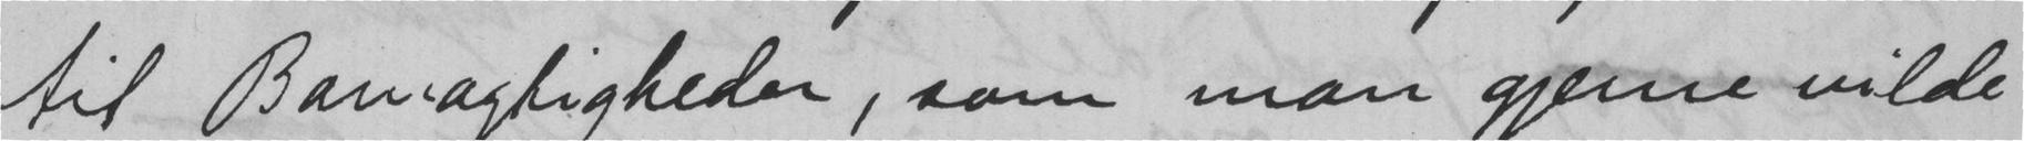til Barnagtigheder, som man gjerne vilde
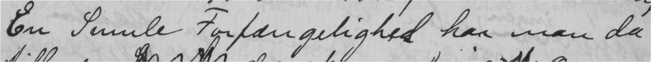En Smule Forfængelighed har man da
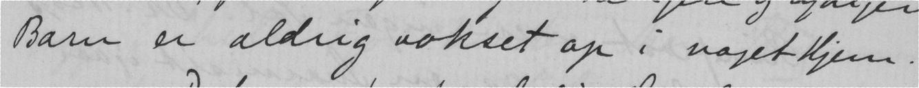Barn er aldrig vokset op i noget Hjem.
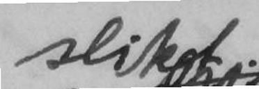slikt
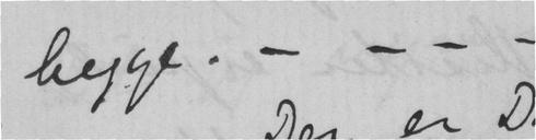begge. — — — —
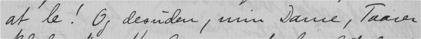at le! Og desuden, min Dame, Taarer
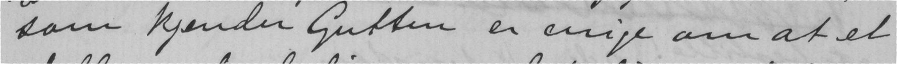som kjender Gutten er enige om at et
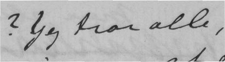? Jeg tror alle,
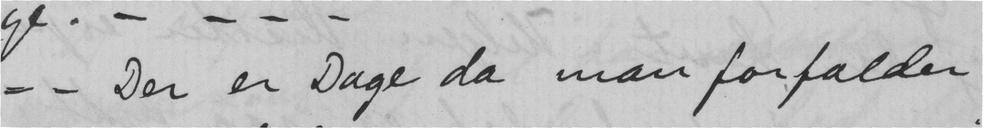- - Der er Dage da man forfalder
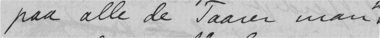paa alle de Taarer man
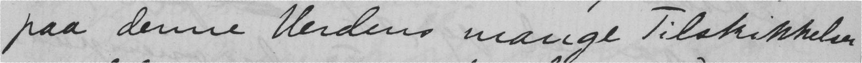paa denne verdens mange Tilskikkelser
